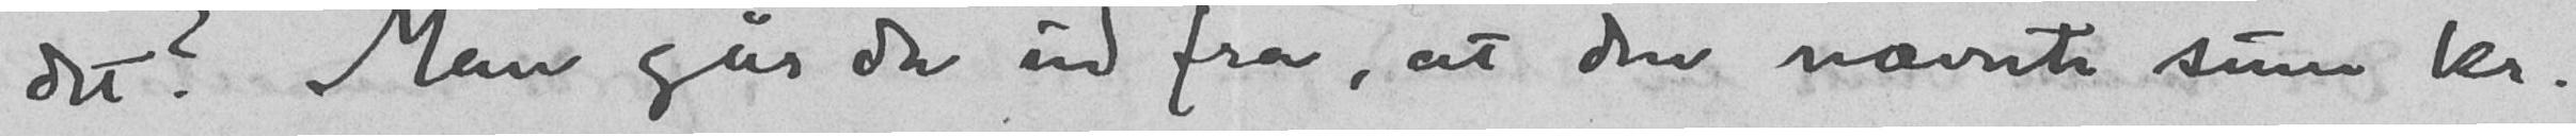det? Man går da ud fra, at den nævnte sum kr.
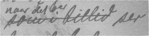som i tillid ser
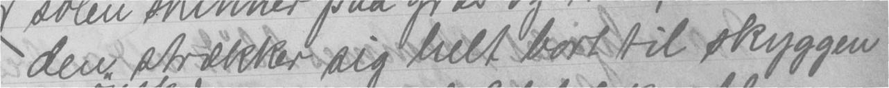den strækker sig helt bort til skyggen
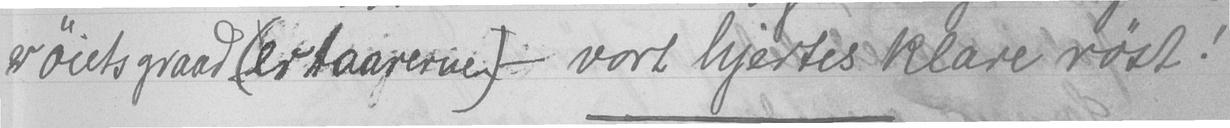er øiets graad (er taarerne) - vort hjertes klare røst!
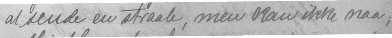at sende en straale, men kan ikke naa;
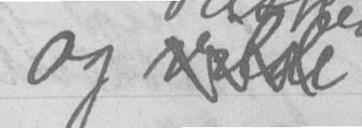og vilde
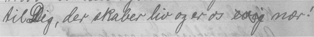til Dig, der skaber liv og er os evig nær!
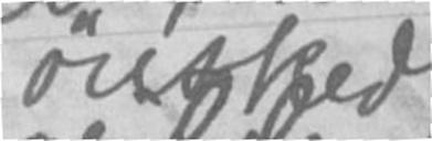ønsked
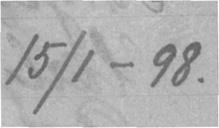15/1 - 98.
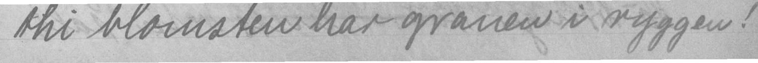thi blomsten har granen i ryggen!
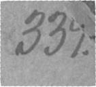339.
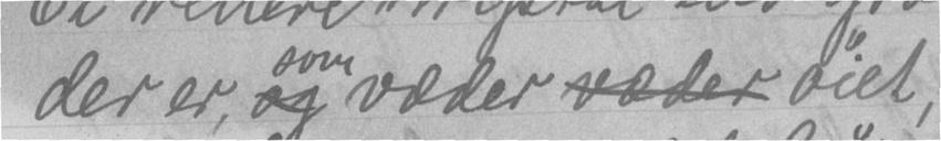der er, ogsom væder væder øiet,
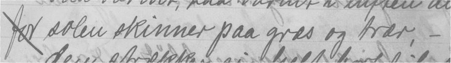for solen skinner paa græs og trær, -
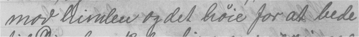mod himlen og det høie for at bede
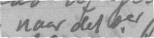naar det ser
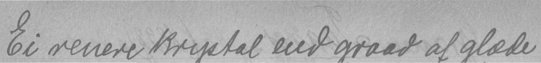Ei renere krystal end graad af glæde,
